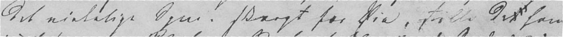det virkelige Spm. skarpt for Øie, stille det ham
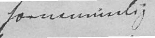fornemmelig
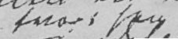hvori han
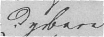dybere
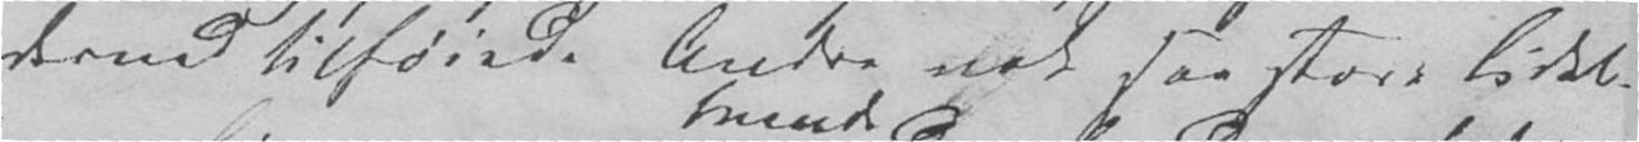derved tilføiede Andre nok saa store Lidel-
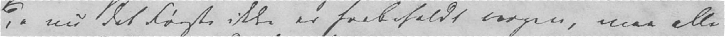da nu det Første ikke er forbeholdt nogen, maa alle
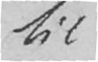til
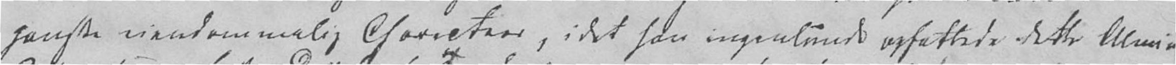ganske eiendommelig Characteer, idet han ingenlunde opfattede dette Almin-
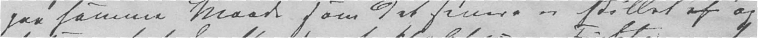paa samme Maade som det senere er stillet af og
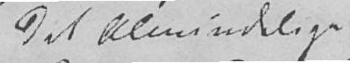det Almindelige
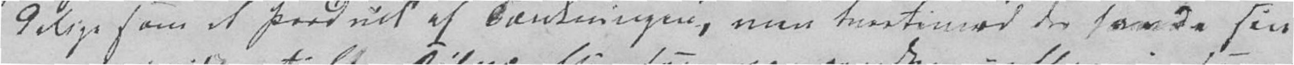delige som et Product af Tænkningen, men tvertimod der svarede sin
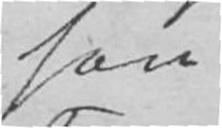han
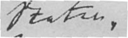Staten.
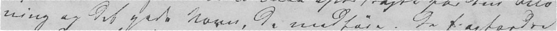ning og det gode Navn, de medføre. De f opfordre
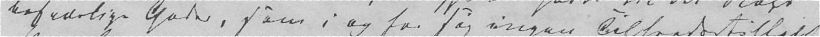besværlige Goder, som i og for sig ingen Tilfredsstillelse
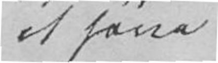at have
